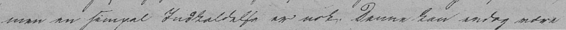men en simpel Indkaldelse er nok. Denne kan endog være
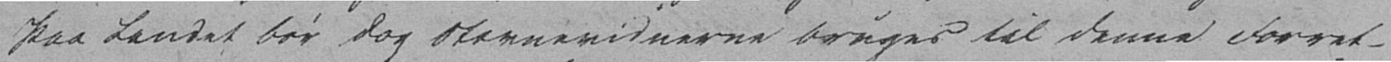Paa Landet bør dog Stevnevidnerne bruges til denne Forret-
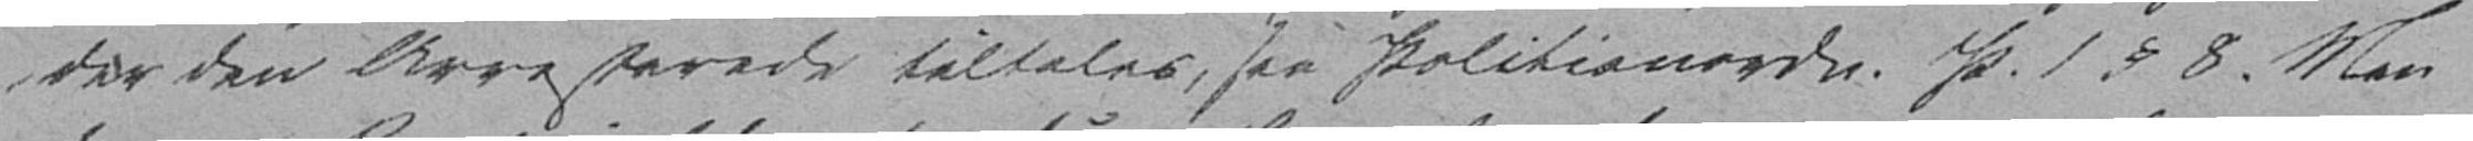der den Arresterede tiltales, see Politianordn. P. 1 § 8. Men
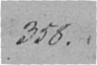358.
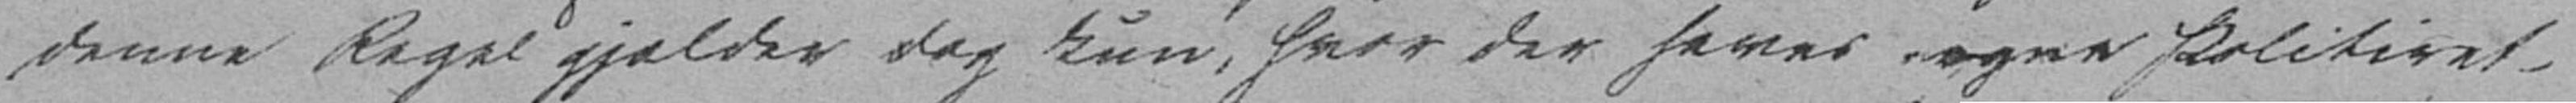denne Regel gjelder dog kun, hvor der haves egne Politiret-
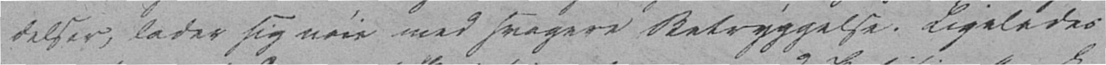delser, lader sig nøie med svagere Betryggelse. Ligeledes
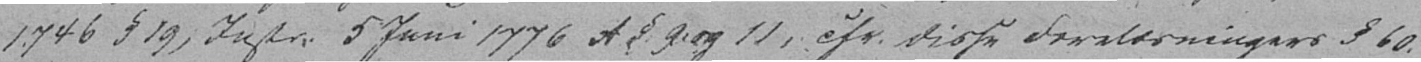1746 § 19, Instr. 5 Juni 1776 A § 9 og 11, cfr. disse Forelæsningers § 60.
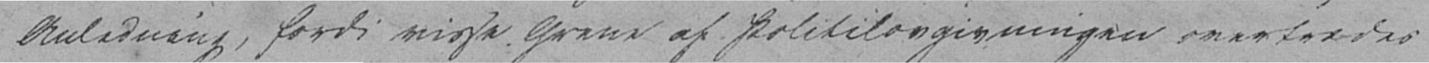Anledning, fordi visse Grene af Politilovgivningen overtrædes
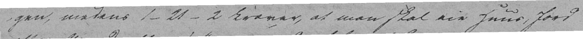gen, medens 1-21-2 kroner, at man skal eie Huus, Jord
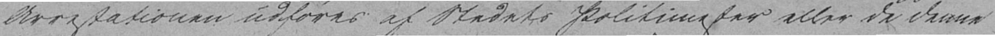Arrestationen udføres af Stedets Politimester eller de denne
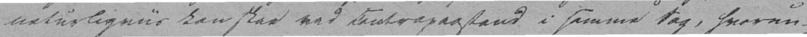naturligviis kan skee ved Contrapaastand i samme Sag, hvorun-
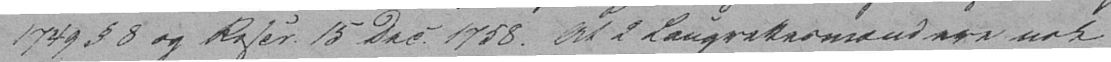1749 § 8 og Rescr. 15 Dec. 1758. At 2 Laugrettesmænd ere nok
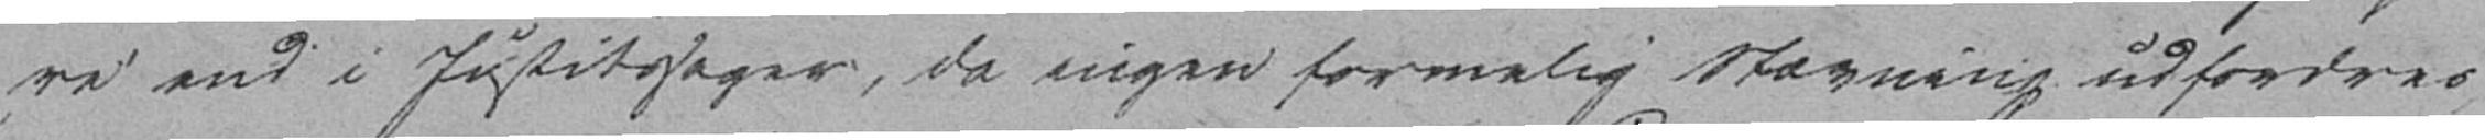re end i Justitssager, da ingen formelig Stevning udfordres,
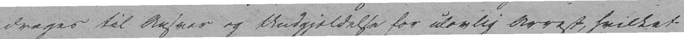drages til Ansvar og Undgjældelse for ulovlig Arrest, hvilket
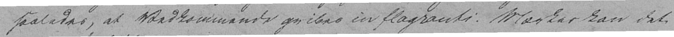saaledes, at Vedkommende gribes in flagranti. Mærkes kan det
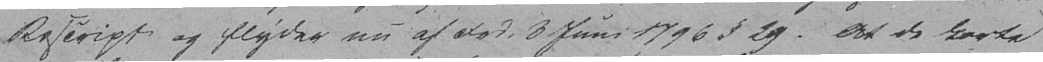Rescript og flyder nu af Frd. 3 Juni 1796 § 29. At de korte
